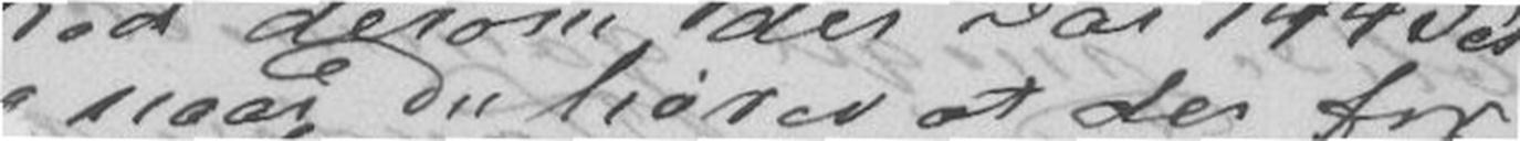naar Du hører at der for
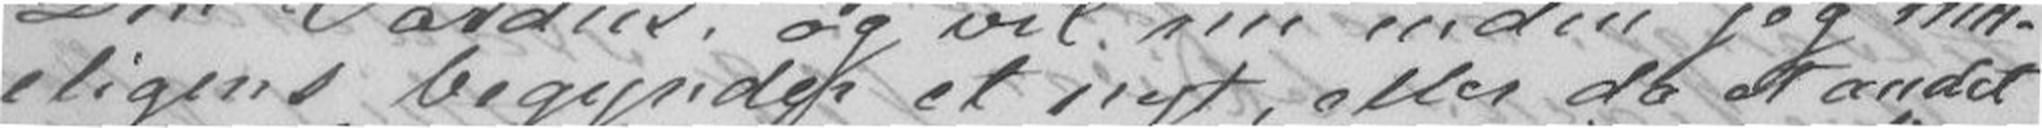eligens begynder et nyt, eller da et andet
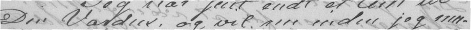Din Vardus, og vil nu inden jeg mu-
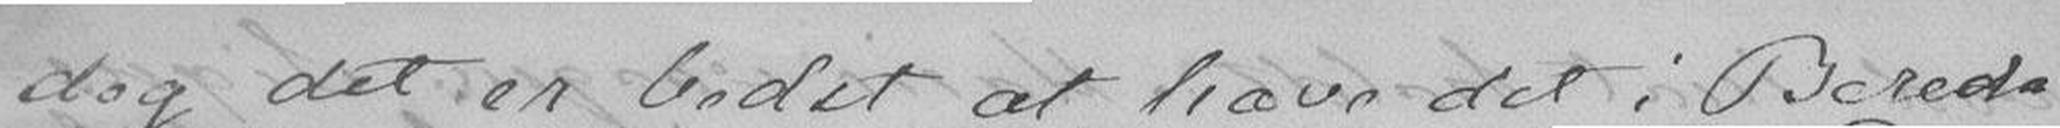dog det er bedst at have det i Bered-
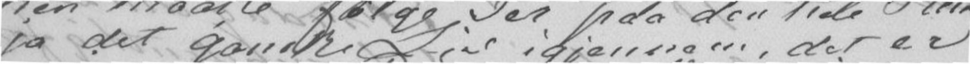ja det ganske Liv igjennem, det er
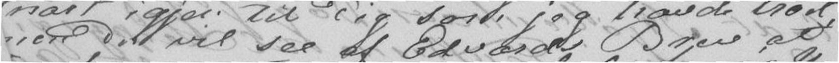men Du vil see af Edvards Brev at
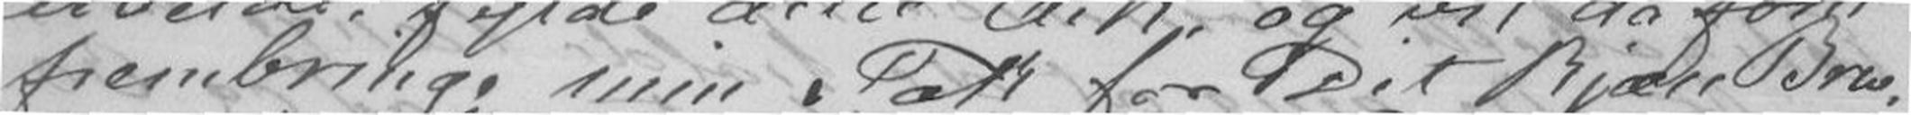frembringe min Tak for Dit kjære Brev,
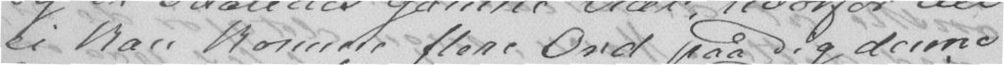ei kan komme flere Ord paa Dig denne
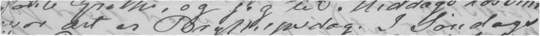hvor det er Bryllupsdag. I Søndags
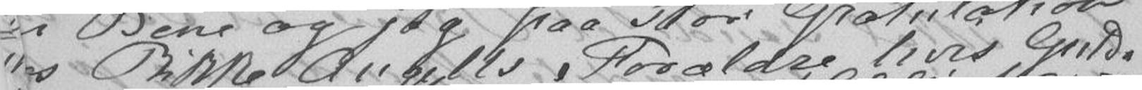hos Rikke Angells Forældre hvis Guld-
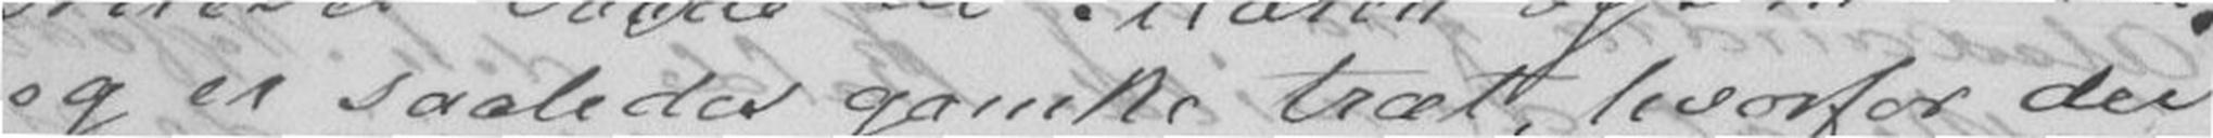og er saaledes ganske træt, hvorfor der
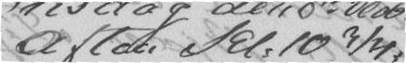Aften Kl.10 3/4.
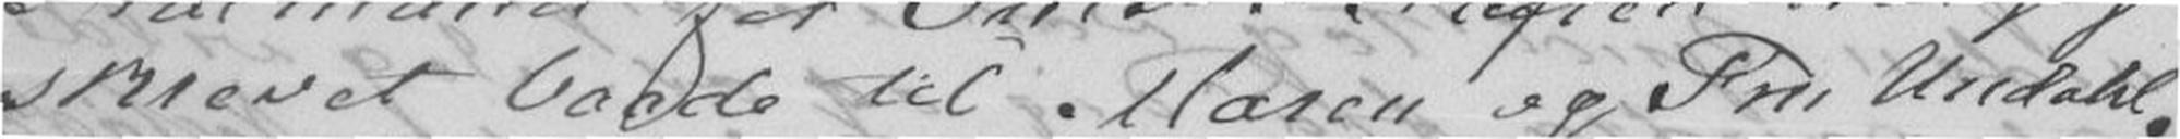skrevet baade til Maren og Fru Undahl,
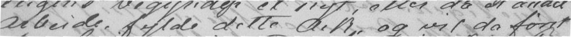Arbeide fylde dette Ark, og vil da først
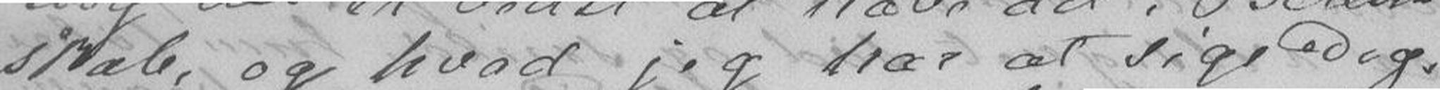skab, og hvad jeg har at sige Dig,
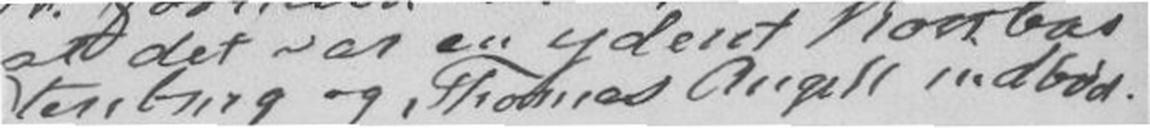tenburg og Thomas Angell indbød.
